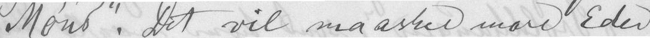Mons. Det vil maaskee more Eder
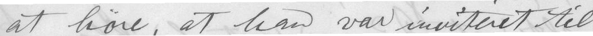at høre, at han var inviteret til
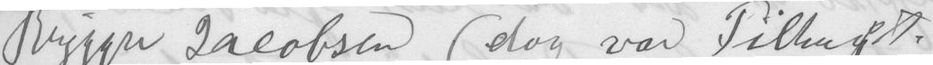Brygger Jacobsen (dog var Tilknyt-
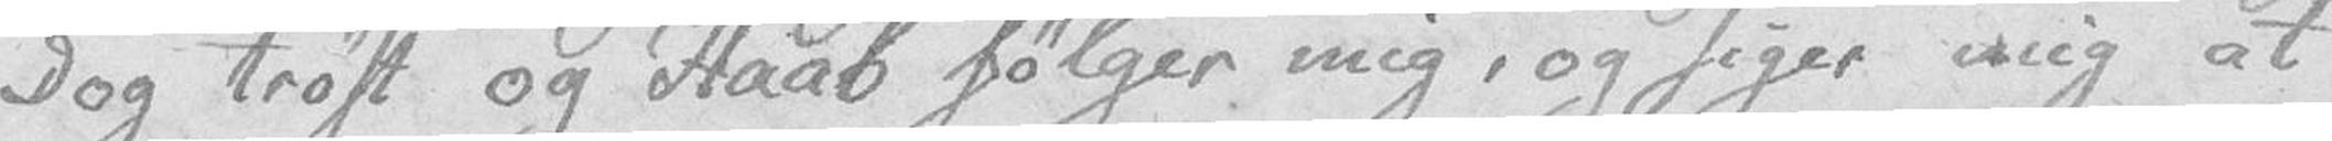Dog trøst og Haab følger mig, og siger mig at
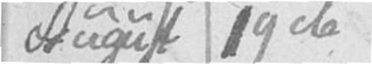August 19de
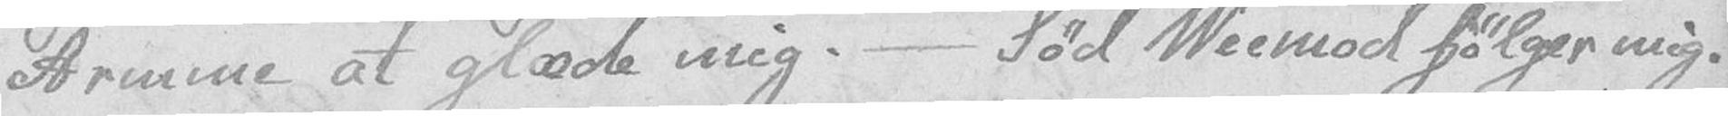Armme at glæde mig. – Sød Veemod følger mig.
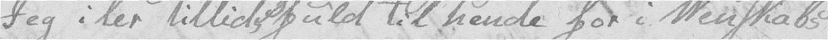Jeg iler tillidsfuld til hende for i Venskabs
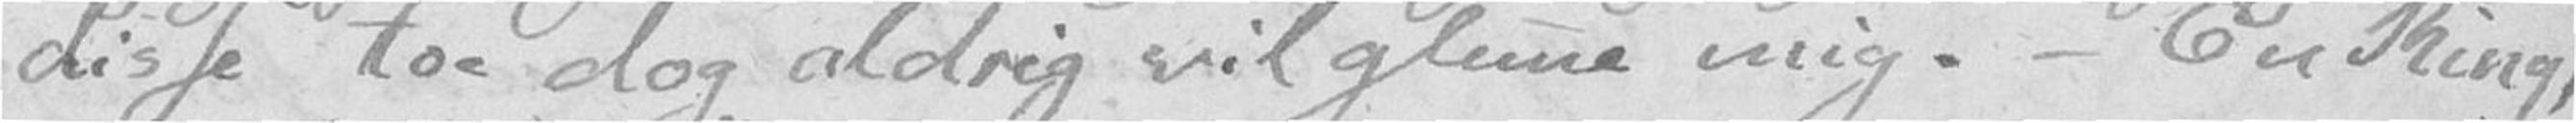disse toe dog aldrig vil glemme mig. – En Ring,
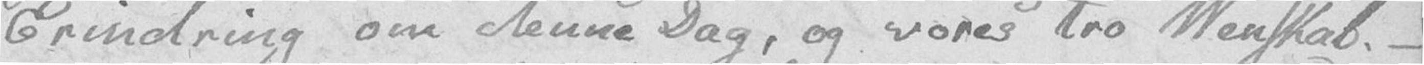Erindring om denne Dag, og vores tro Venskab. –
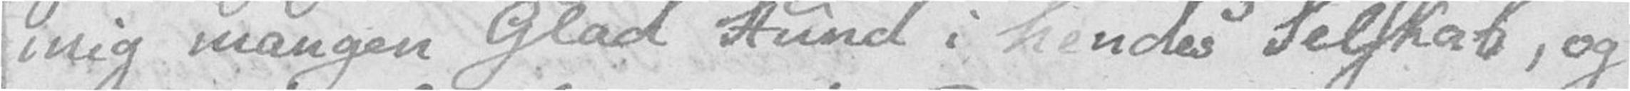mig mangen Glad Stund i hendes Selskab, og
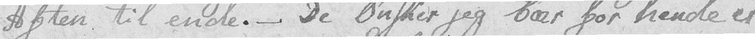Aften til ende. – De Ønsker jeg bær for hende er
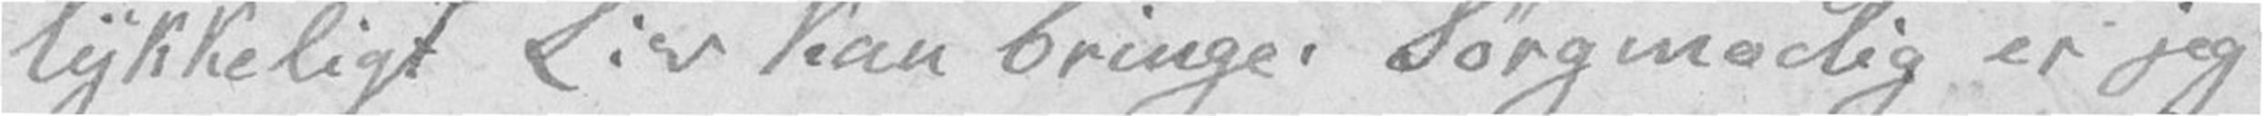lykkeligt Liv kan bringe. Sørgmodig er jeg
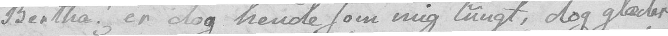Bertha! er dog hende som mig tungt, dog glæder
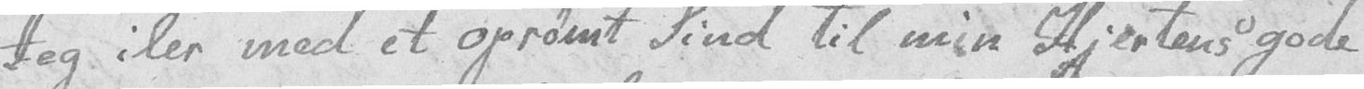Jeg iler med et oprømt Sind til min Hjertens gode
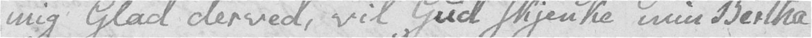mig Glad derved, vil Gud skjenke min Bertha
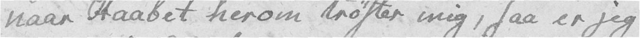naar Haabet herom trøster mig, saa er jeg
